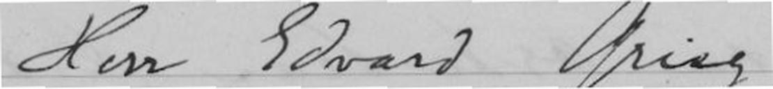Herr Edvard Grieg
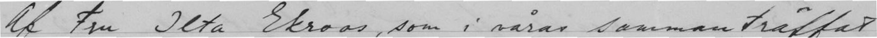Af Fru Ilta Ekroos, som i våras sammanträffat
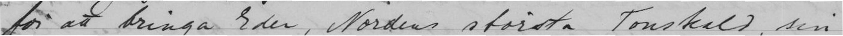för att bringa Eder, Nordens största Tonskald, sin
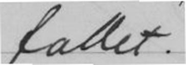fallet.
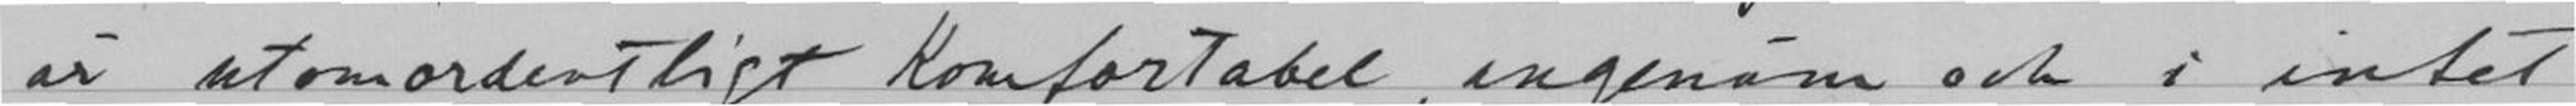är utomordentligt komfortabel, ingenäm och i intet
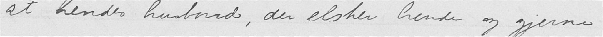at hendes husbond, der elsker hende og gjerne
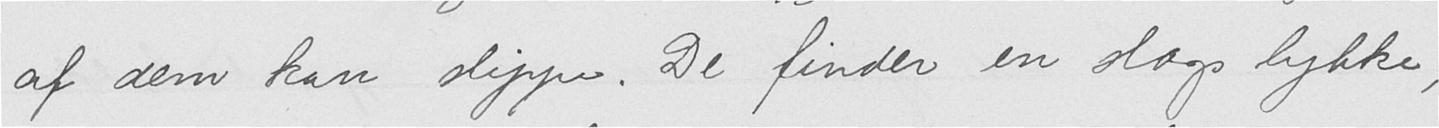af dem kan slippe. De finder en slags lykke,
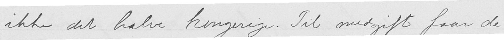ikke det halve kongerige. Til medgift faar de
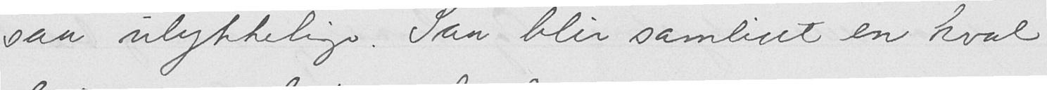saa ulykkelige. Saa blir samlivet en kval,
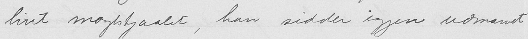livet magtstjaalet, han sidder igjen udmattet,
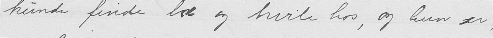kunde finde læ og hvile hos, og hun ser,
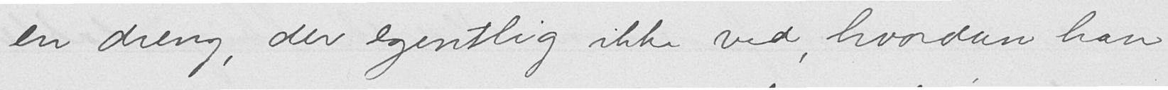en dreng, der egentlig ikke ved, hvordan han
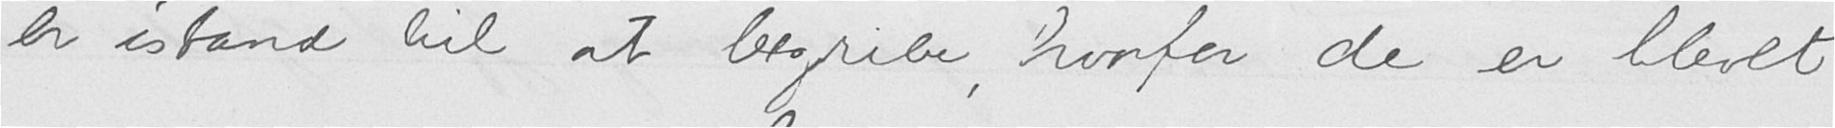er istand til at begribe, hvorfor de er blevet
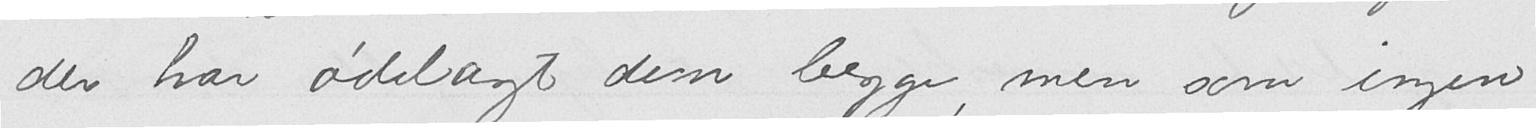der har ødelagt dem begge, men som ingen
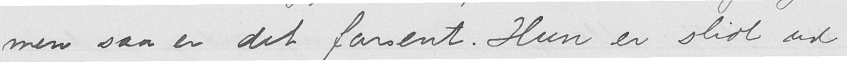men saa er det forsent. Hun er slidt ud
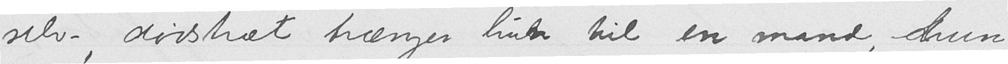selv, dødstræt længes hun til en mand, hun
